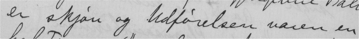er skjøn og Udførelsen varer en
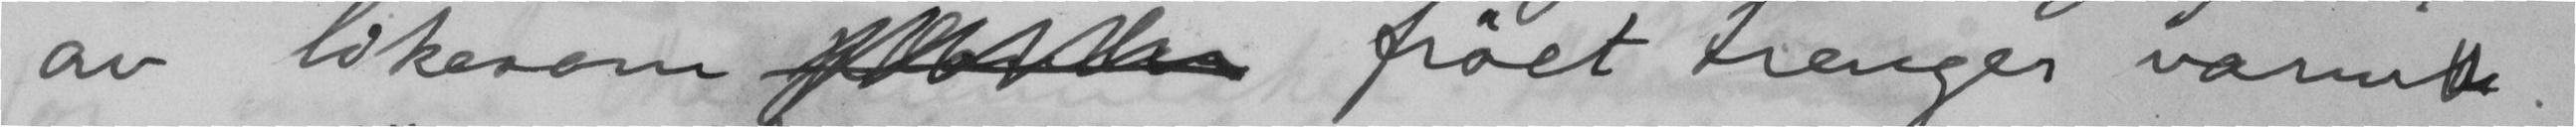av likesom  frøet trenger varme
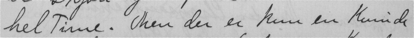hel Time. Men der er kun en Kvinde
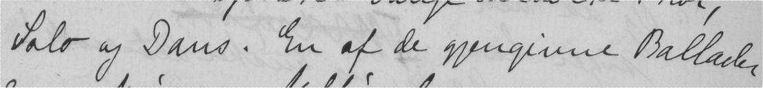Solo og Dans. En af de gjengivne Ballader
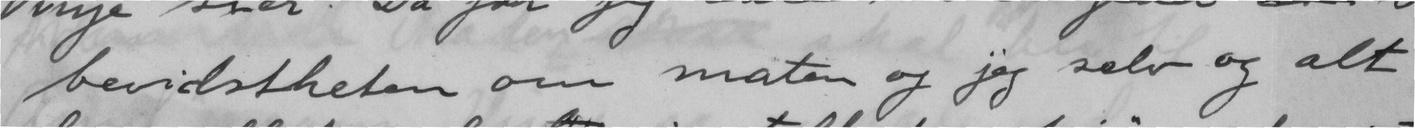bevidstheten om maten og jeg selv og alt
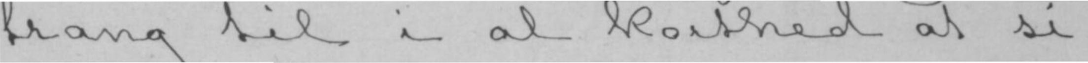trang til i al korthed at si-
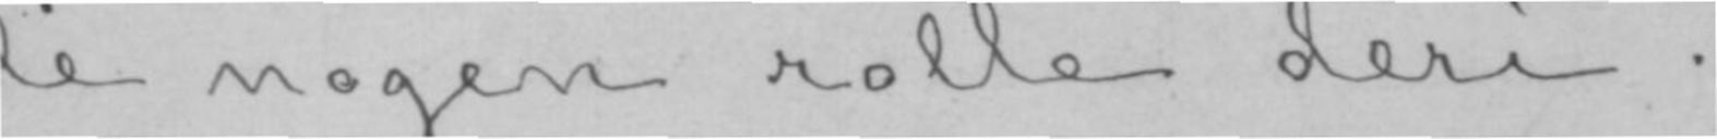le nogen rolle deri.
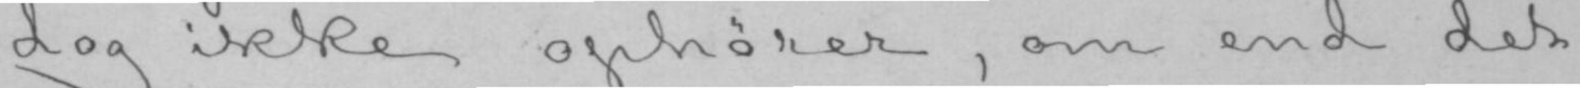dog ikke ophører, om end det
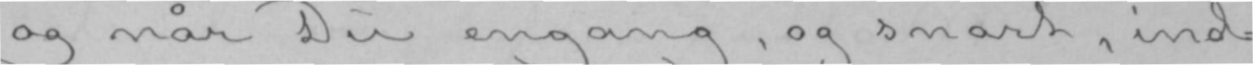og når Du engang, og snart, ind-
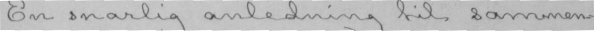En snarlig anledning til sammen-
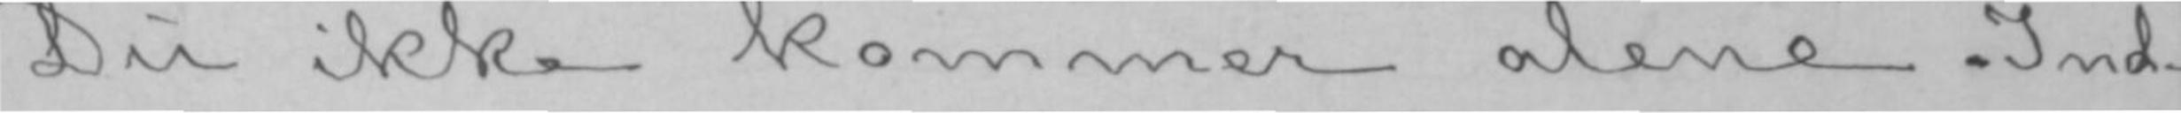Du ikke kommer alene. Ind-
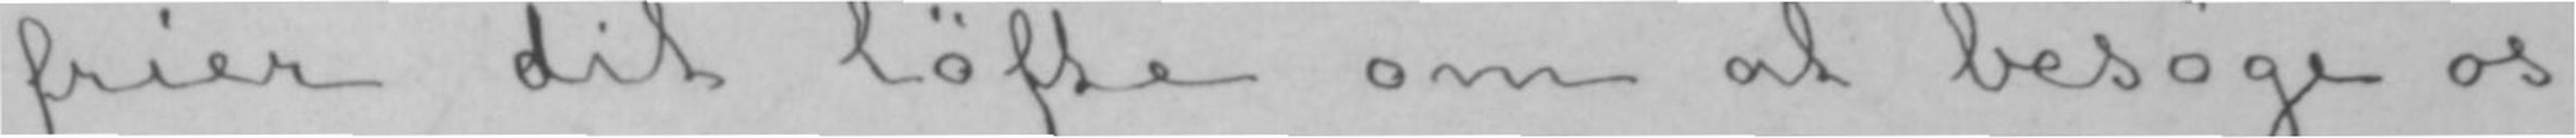frier dit løfte om at besøge os
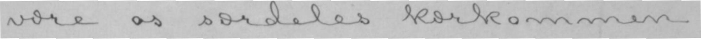være os særdeles kærkommen,
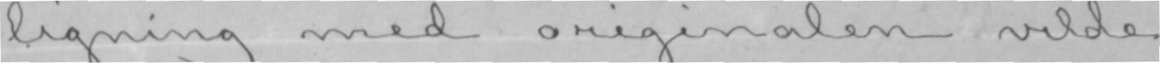ligning med originalen vilde
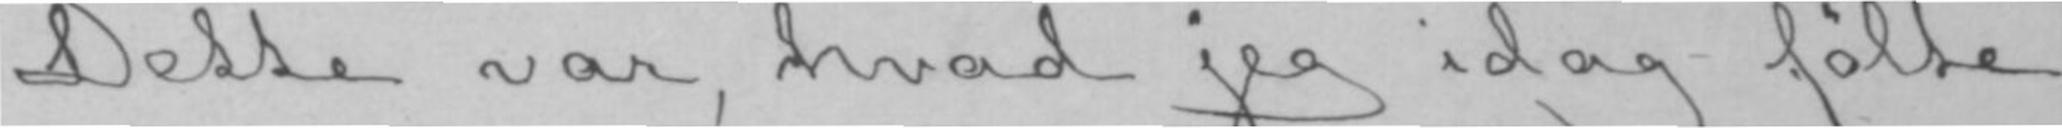Dette var, hvad jeg idag følte
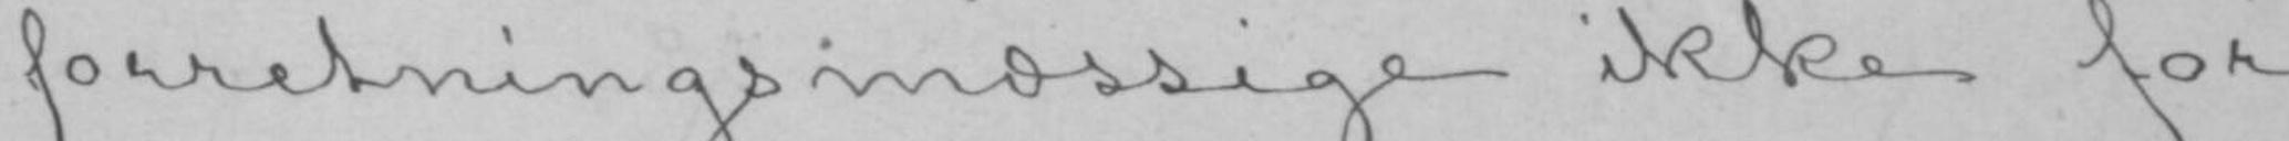forretningsmæssige ikke for
